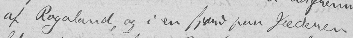af Rogaland, og i en fjord paa Jæderen
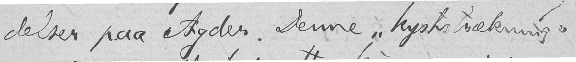delser paa Agder. Denne "kyststrækning"
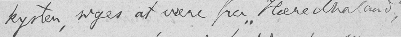kyster, siges at være fra "Hæredhaland",
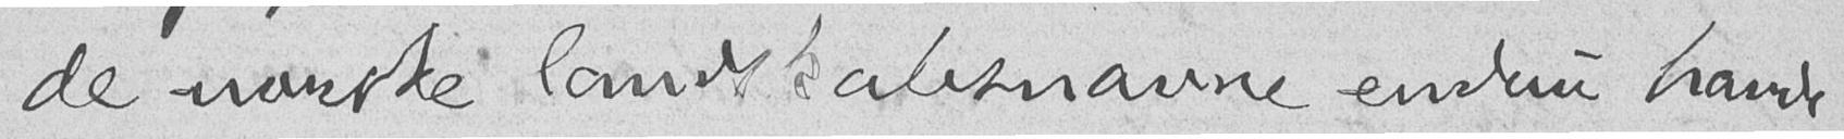de norske landskabsnavne endnu havde
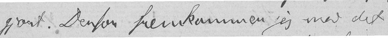gjort. Derfor fremkommer jeg med det
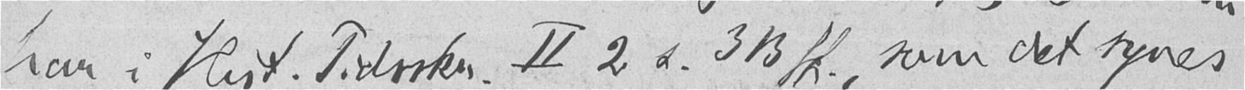har i Hist. Tidsskr. II 2 s. 313 ff., som det synes
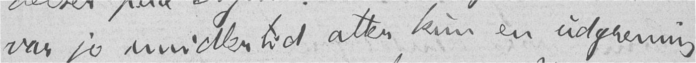var jo imidlertid atter kun en udgrening
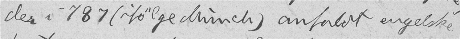der i 787 (ifølge Munch) anfaldt engelske
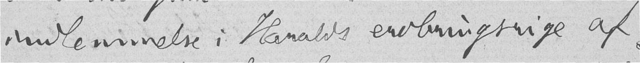indlemmelse i Haralds erobringsrige af-
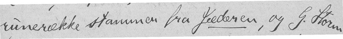runerække stammer fra Jæderen, og G. Storm
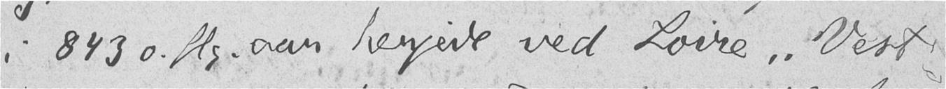i 843 o. flg. aar herjede ved Loire "Vest-
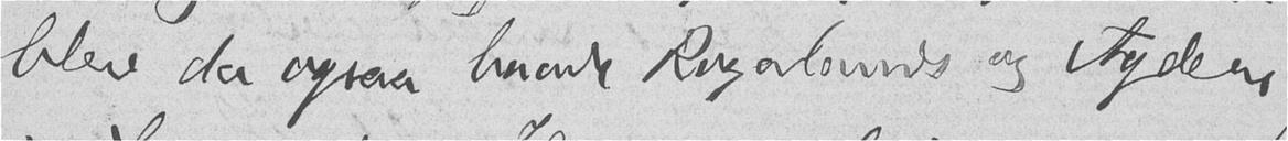blev da ogsaa baade Rogalands og Agders
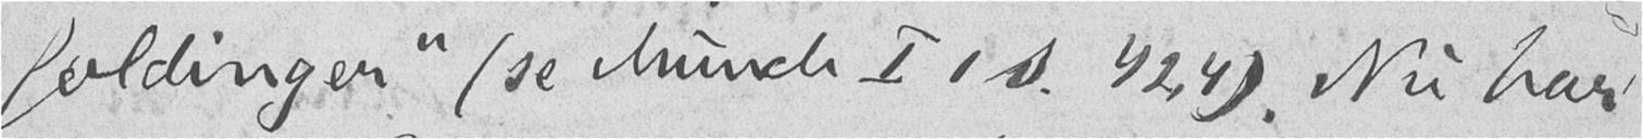foldinger" (se Munch I 1 s. 424). Nu har
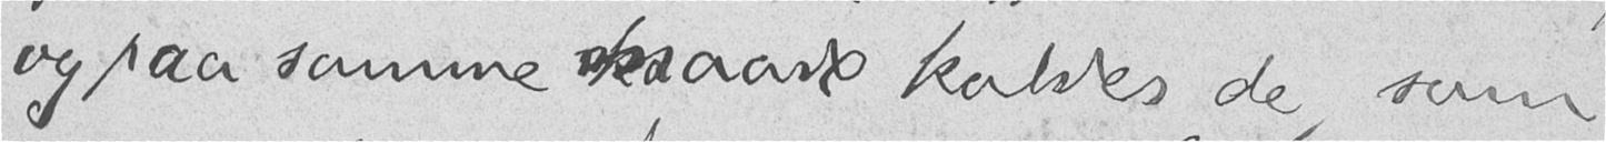og paa samme maade kaldes de, som
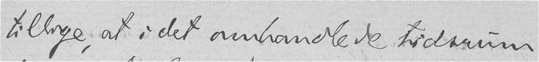tillige, at i det omhandlede tidsrum
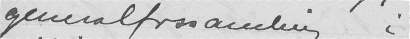generalforsamling i
